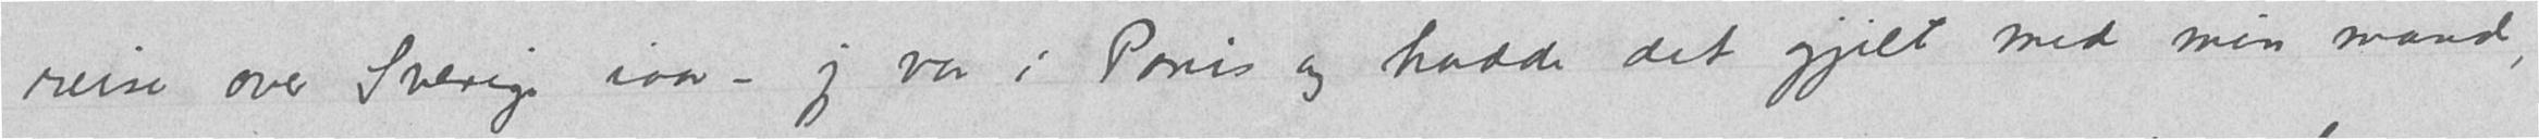reise over Sverige iaar - jeg var i Paris og hadde det gjilt med min mand,
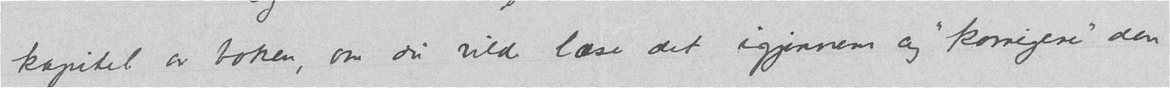kapitel av boken, om du vilde læse det igjennem og "korrigere" den
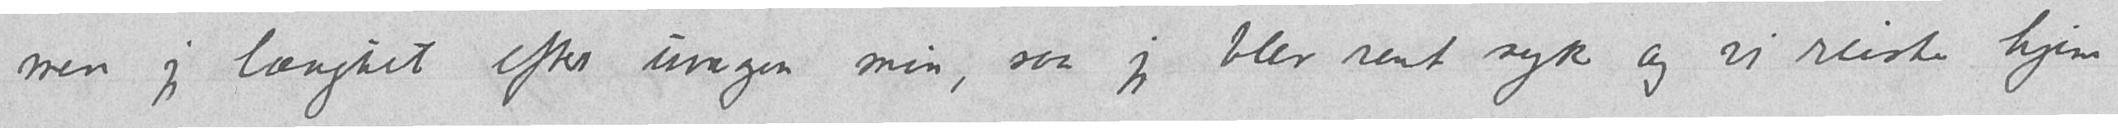men jeg længtet efter ungen min, saa jeg blev rent syk og vi reiste hjem
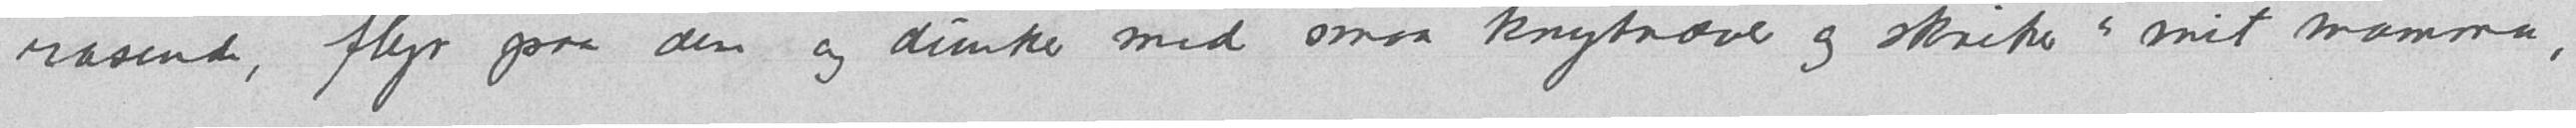rasende, flyr paa dem og dunker med smaa knyttnæve og skriker "mit mamma,
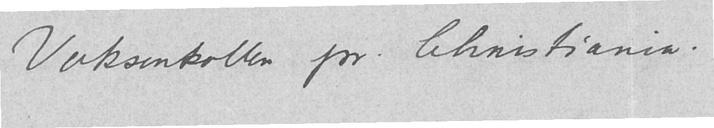Voksenkollen pr. Christiania.
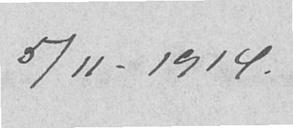5/11-1914.
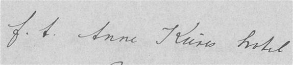f.t.  Anne Kures hotel
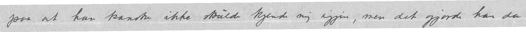paa at han kanske ikke skulde kjende mig igjen, men det gjorde han da
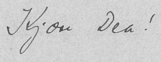Kjære Dea!
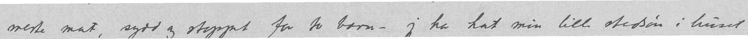meste mat, sydd og stoppet for to barn - jeg har hat min lille stedsøn i huset
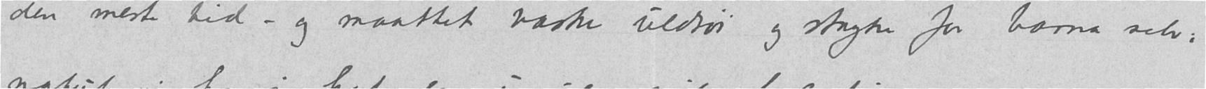den meste tid - og maattet vaske uldtøi og stryke for barna selv,
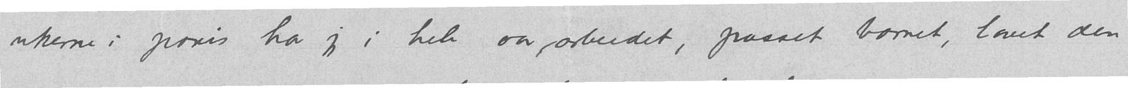ukerne i paris har jeg i hele aar arbeidet, passet barnet, lavet den
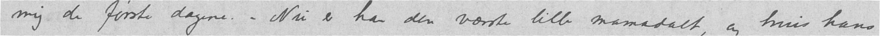mig de første dagene. - Nu er han den værste lille mamadalt, og hvis hans
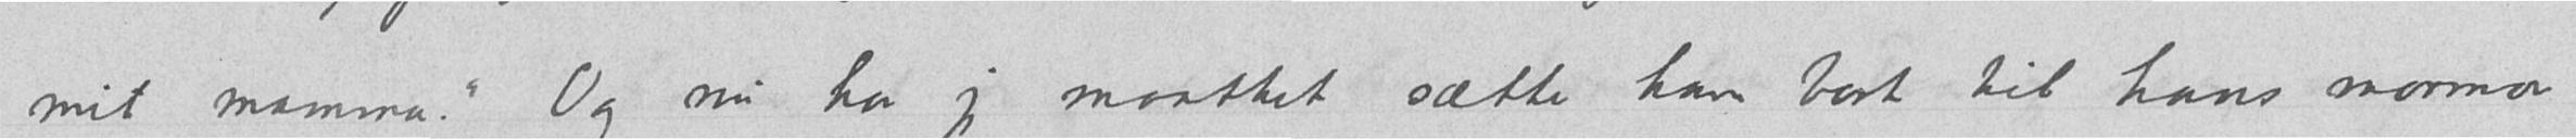mit mamma." Og nu har jeg maattet sætte han bort til hans mormor
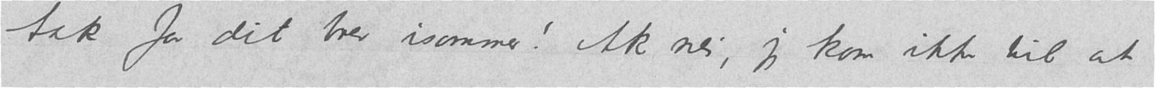tak for dit brev isommer! Ak nej, jeg kom ikke til at
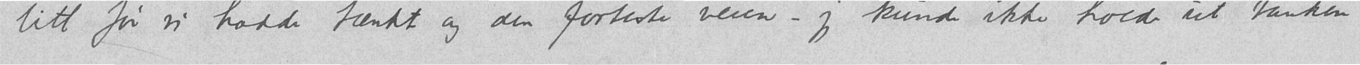litt før vi hadde tænkt og den forteste veien - jeg kunde ikke holde ud tanken
